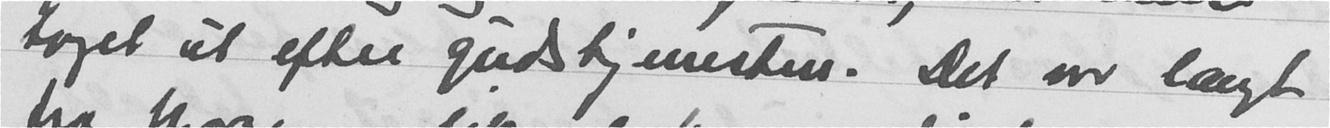toget ut efter gudstjeneste. Det var langt
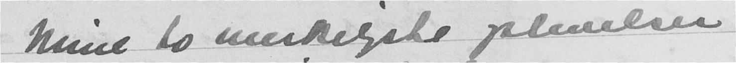Mine to merkeligste oplevelser
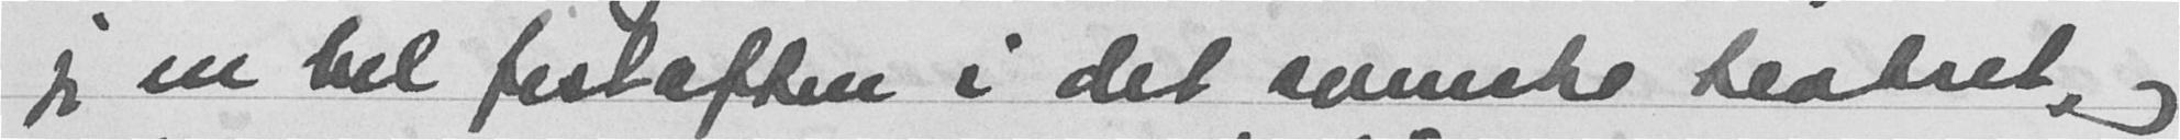meg en hel festaften i det svenske teatret, og
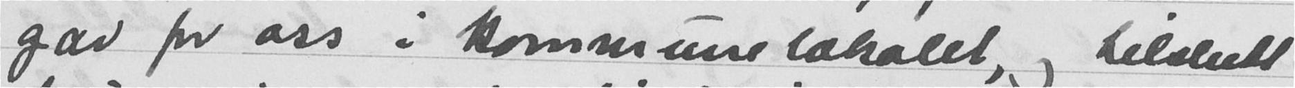gav for oss i kommunelokalet, og tilslutt
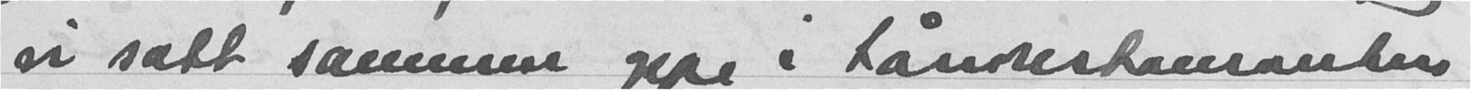vi satt sammen oppe i tårnsestamanten
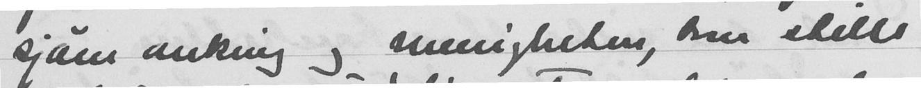sjøen anking og menigheten, som stille
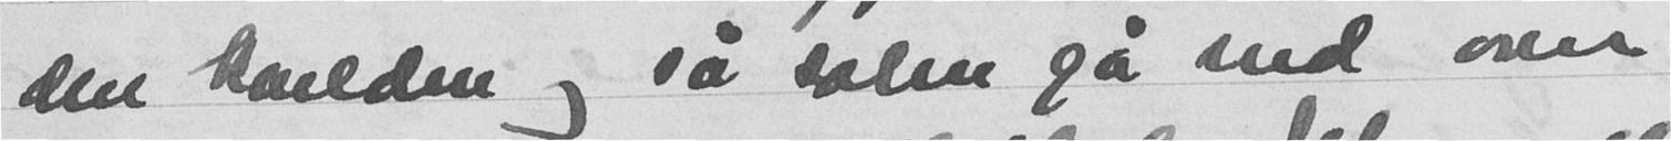den kvelden og så salen gå ind over
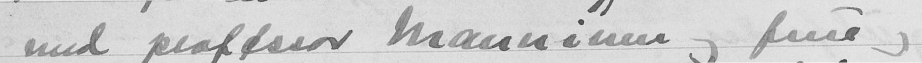med professor Manninne og frue og
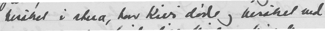besøket i stua, hvor Kiers døde og beriket ved
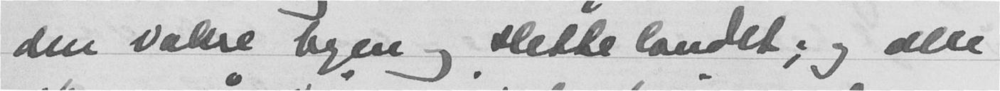den vakre byen og sette landet; og alle
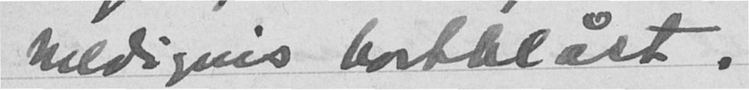heldigvis bortblåst.
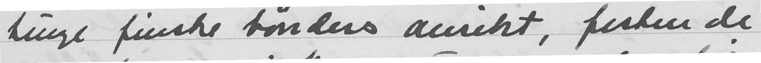tunge finske bønders ansikt, fisken de
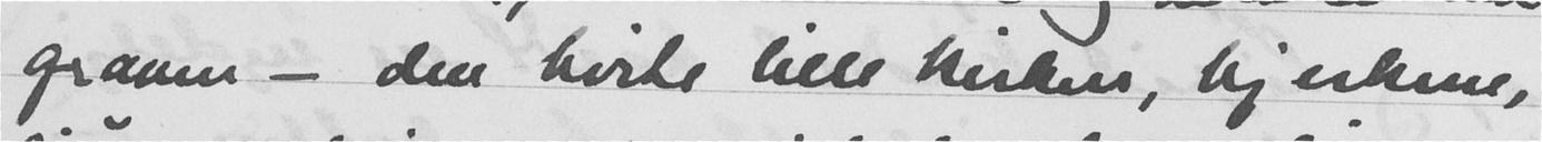graven - den hvite lille kisten, hjertene,
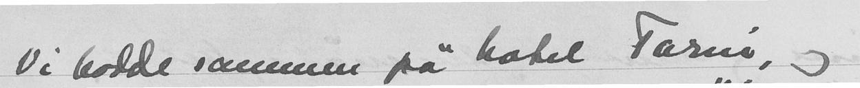Vi bodde sammen på hotel Farsi, og
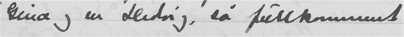Gina og en Hedvig, så fullkomment
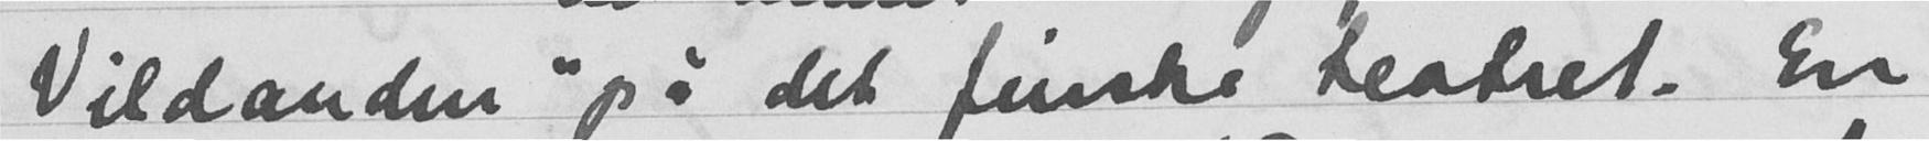Vildanden på det finske teatret. En
